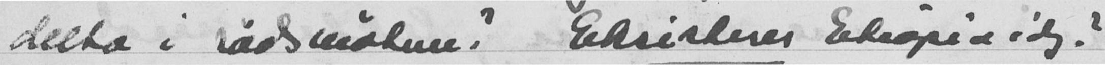delta i rådsmøtene. Eksisterer Etiopia idag?
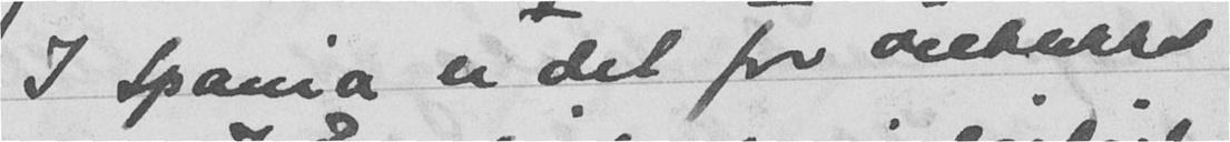I Spania er det for øieblikket
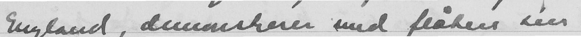England, demonstrerer med flåten sin
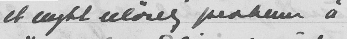et nytt uløslig problem å
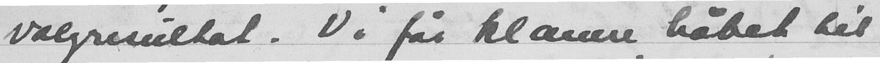valgresultat. Vi får klamre håbet til
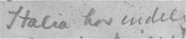Italia har endelig
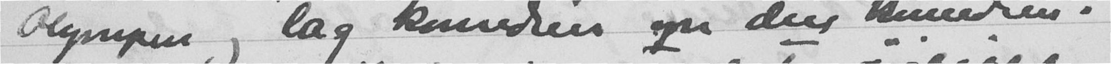Olympen, lag komedier også den komedien!
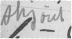skjold
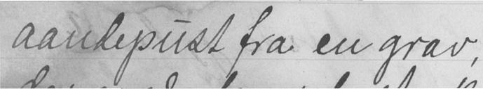aandepust fra en grav,
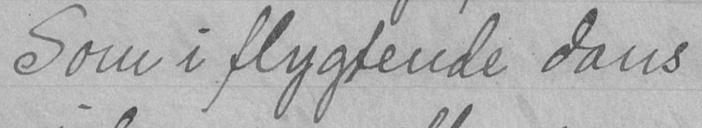Som i flygtende dans
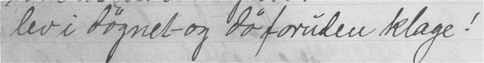lev i døgnet og dø foruden klage!
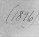(1896)
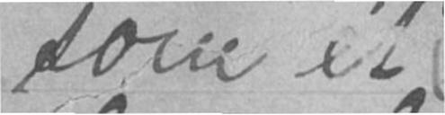som et
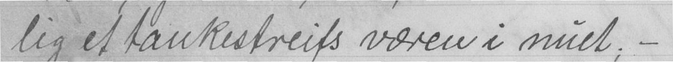lig et tankestreifs væren i nuet; -
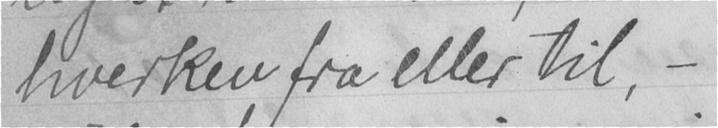hverken fra eller til, -
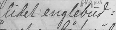lidet englebud:
